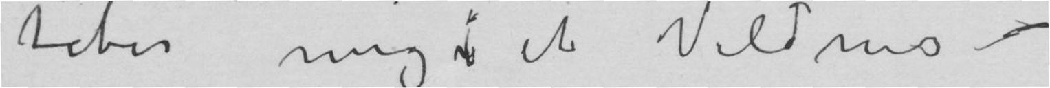taber mig i et vildnis –
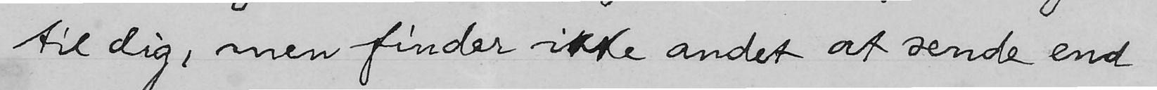til dig, men finder ikke andet at sende end
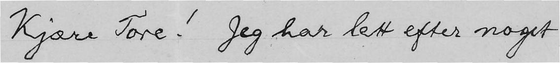Kjære Tore! Jeg har lett efter noget
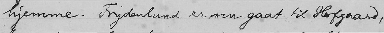hjemme. Frydenlund er nu gaat til Hofgaard,
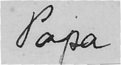Papa
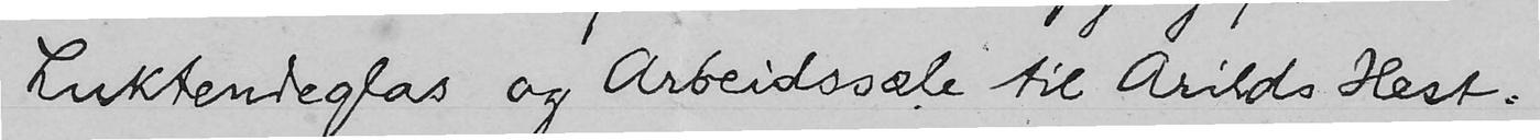Luktendeglas og Arbeidssæle til Arilds Hest.
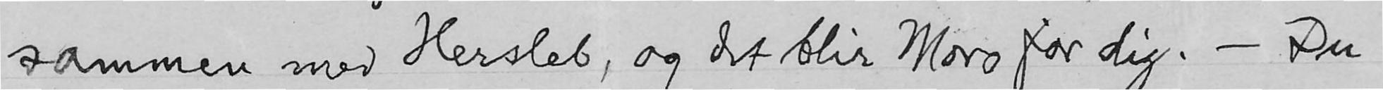sammen med Hersleb, og det blir Moro for dig. - Du
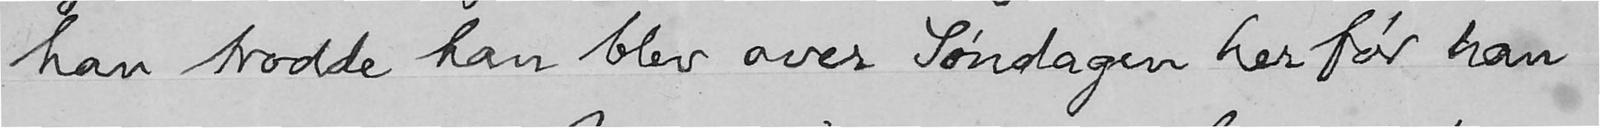han trodde kan blev over Søndagen her før han
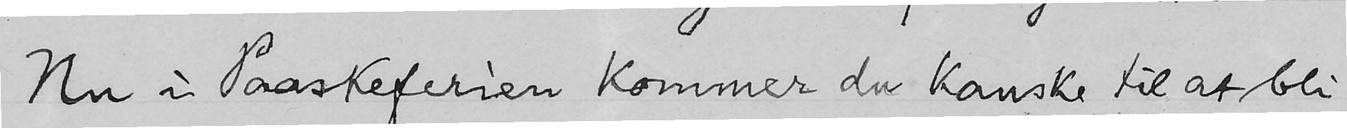Nu i Paaskeferien kommer du kanske til at bli
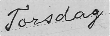Torsdag
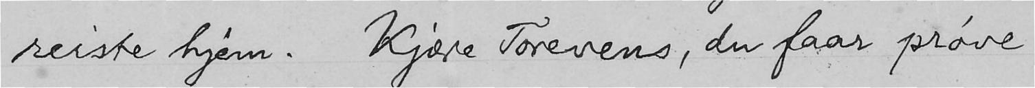reiste hjem. Kjære Torevens, du faar prøve
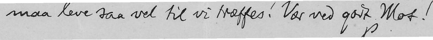maa leve saa vel til vi træffes! Vær ved godt Mot!
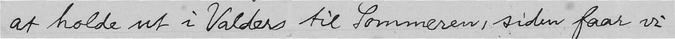at holde ut i Valders til Sommeren, siden faar vi
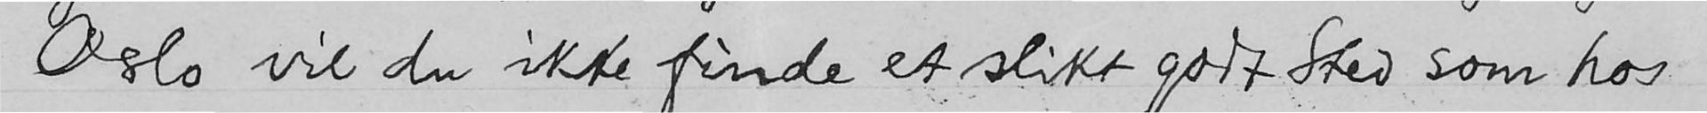Oslo vil du ikke finde et slikt godt Sted som hos
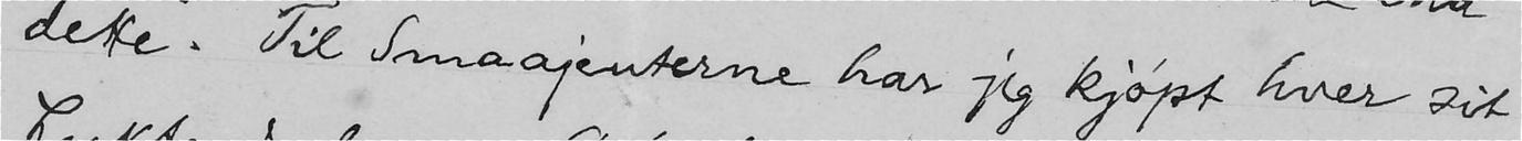dette. Til Smaajenterne har jeg kjøpt hver sit
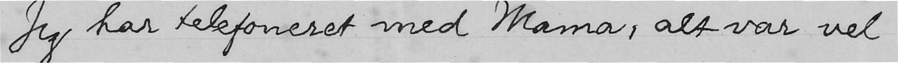Jeg har telefoneret med Mama, alt var vel
